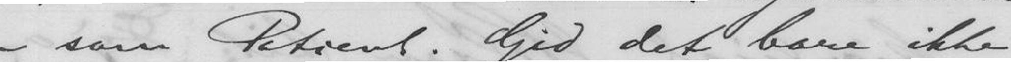- som Patient. Gid det bare ikke
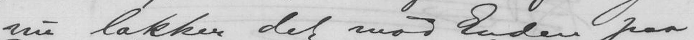nu lakker det mod Enden paa
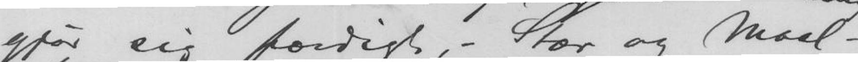gjør sig færdigt, - Stær og Maal-
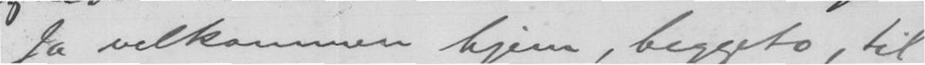Ja velkommen hjem, beggeto, til
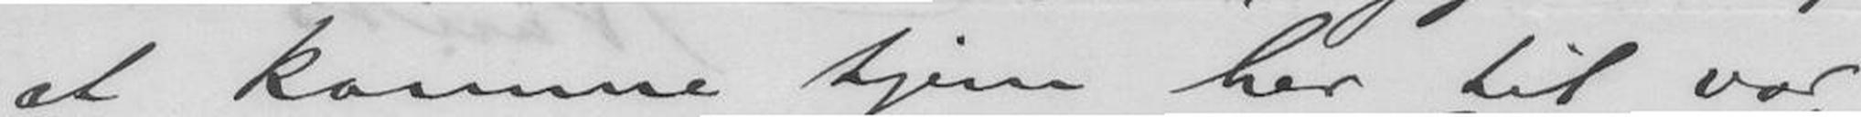at komme hjem her til vor
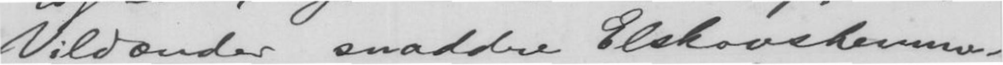Vildænder snaddre Elskovshemme-
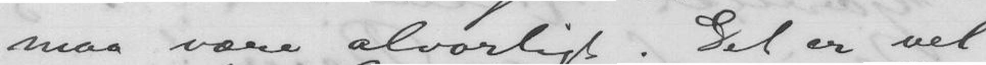maa være alvorligt. Det er vel
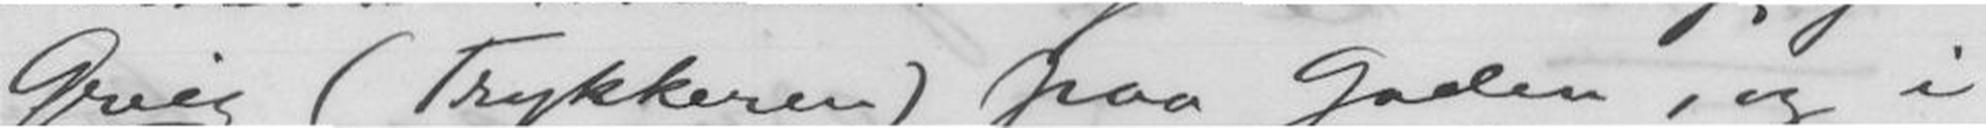Grieg (Trykkeren) paa Gaden, og i
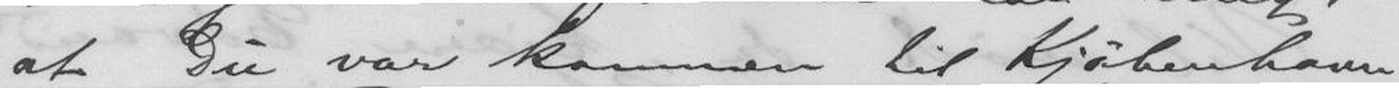at Du var kommen til Kjøbenhavn
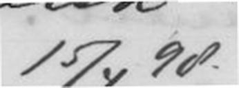15/4 98
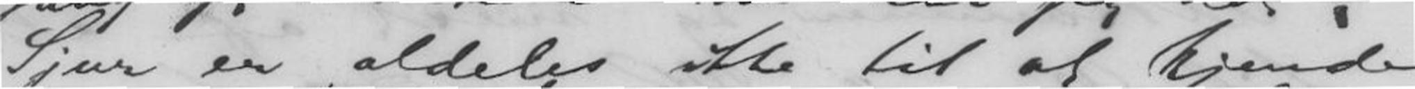Sjur er aldeles ikke til at kjende
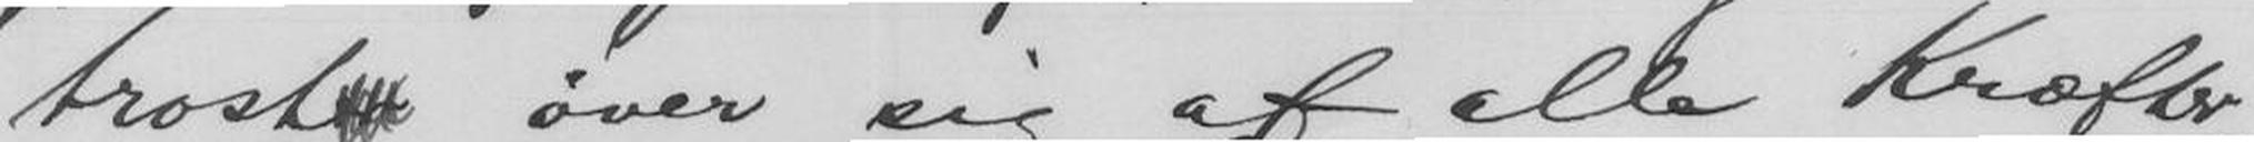trost øver sig af alle Kræfter,
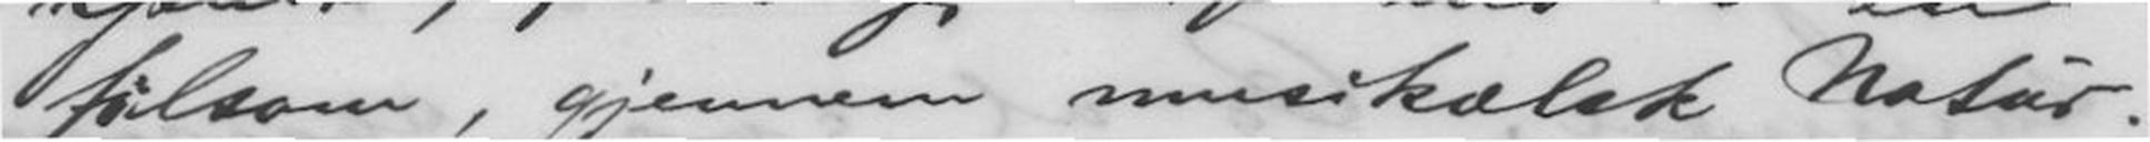følsom, gjennem musikalsk Natur.
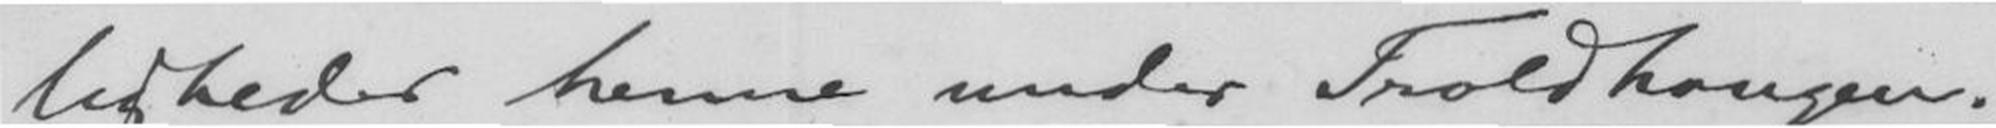ligheder henne under Troldhaugen.
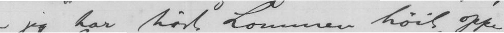- jeg har hørt Lommen Høit oppe
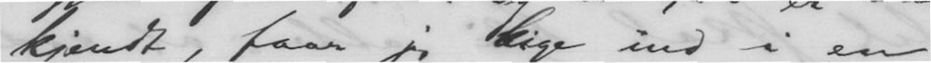kjendt, faar jeg kige ind i en
